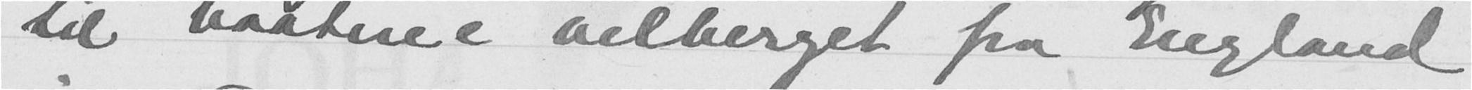til baatene velberget fra England
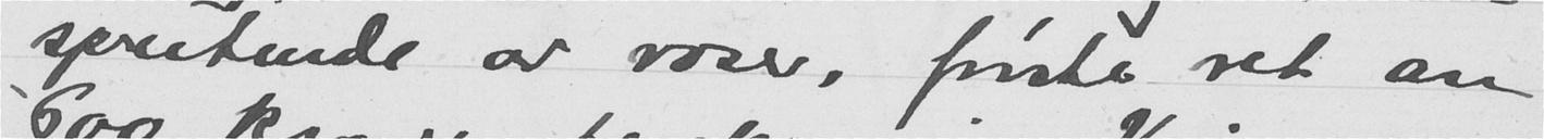sprutende av roser, første ret en
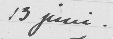13 juni.
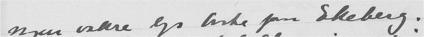nogen vakre lys borte paa Ekeberg.
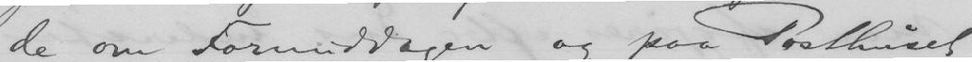de om Formiddagen og paa Posthuset
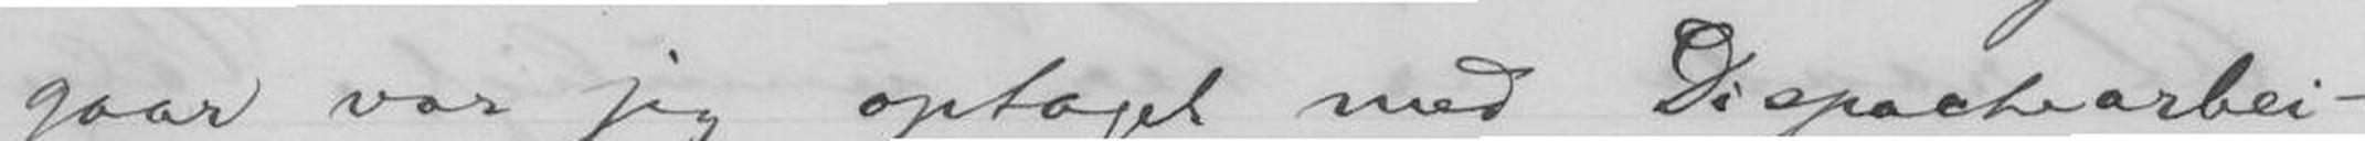gaar var jeg optaget med Dispachearbei-
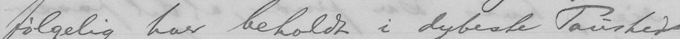følgelig har beholdt i dybeste Taushed
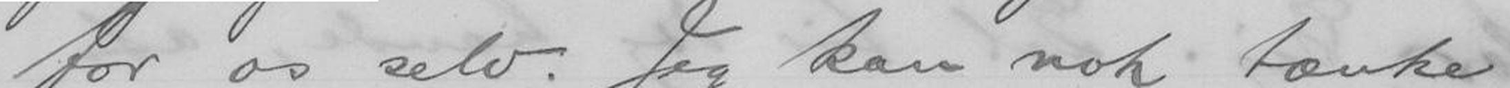for os selv. Jeg kan nok tænke
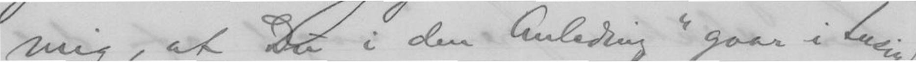mig, at Du i den Anledning "gaar i tusind
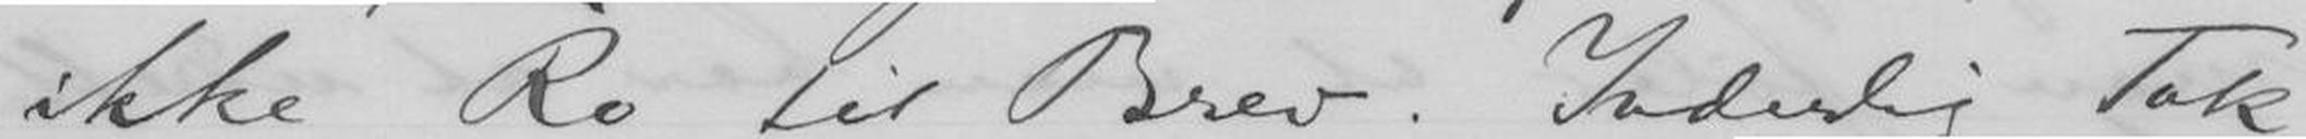ikke Ro til Brev. Inderlig Tak
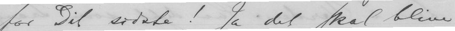for Dit sidste! Ja det skal blive
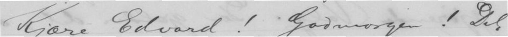Kjære Edvard! Godmorgen! Det
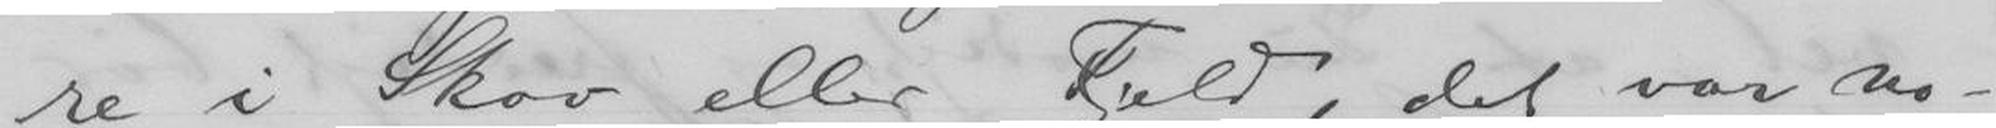re i Skov eller Fjeld, det var no-
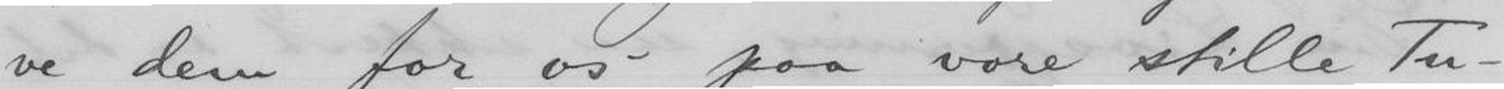ve dem for os paa vore stille Tu-
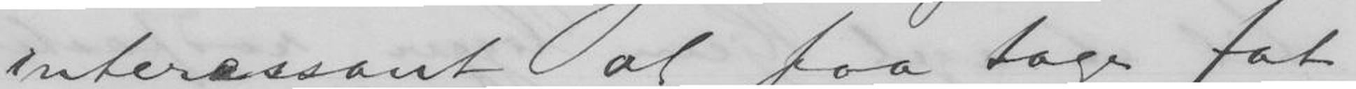interæssant at faa tage fat
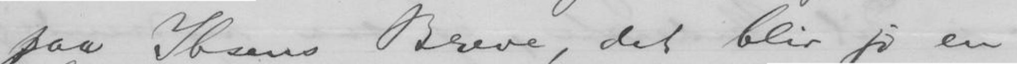paa Ibsens Breve, det blir jo en
